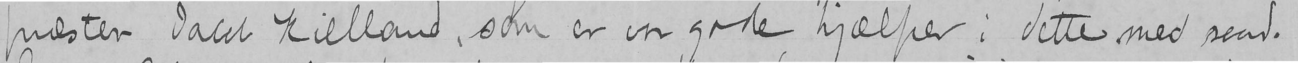præsten Jacob Kielland, som er en gode hjælper i dette med raad.
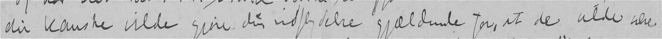du kanske vilde gjøre din indflydelse gjældende for, at de vilde være
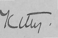Kitty.
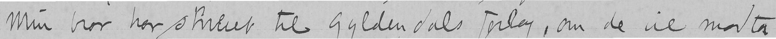Min bror har skrevet til Gyldendals forlag, om de vil modta
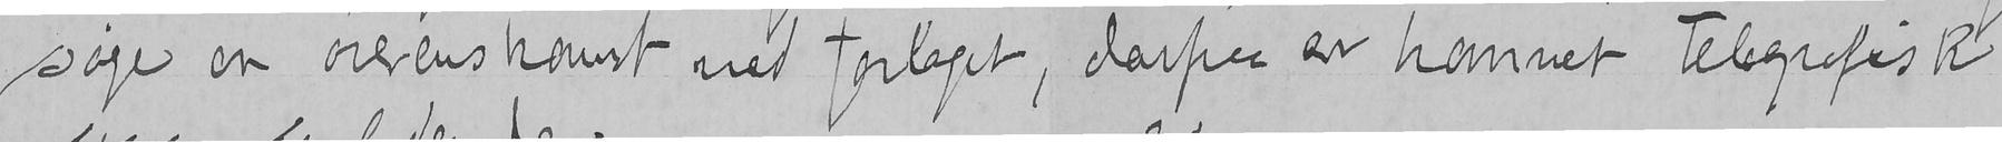søge en overenskomst med forlaget, derpaa er kommet telegrafisk
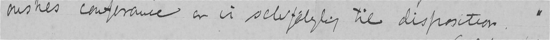ønskes conferance er vi selvfølgelig til disposition."
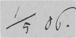1/5 06.
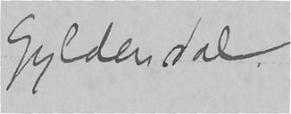Gyldendal.
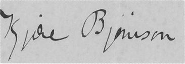Kjære Bjørnson
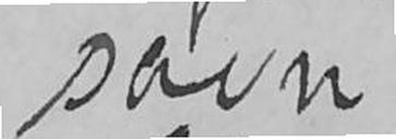savn
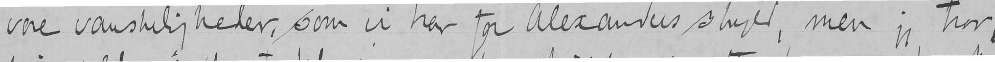vore vanskeligheder, som vi har for Alexanders skyld, men jeg tror,
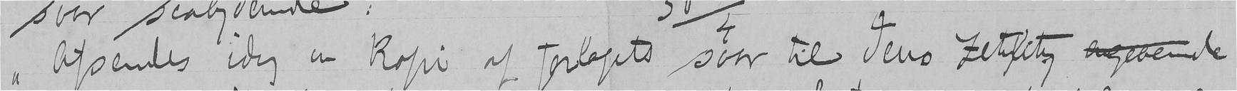"Afsendes idag en kopi af forlagets svar til Jens Zetlitz angaaende
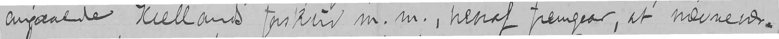angaaede Kiellands forskud m.m. heraf fremgaar, at nævnevær-
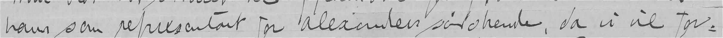ham som representant for Alexanders sødskende, da vi vil for-
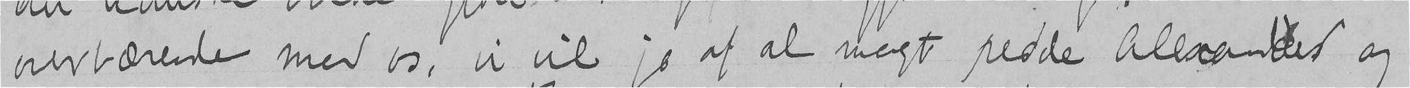overbærende med os, vi vil jo af al magt redde Alexander og
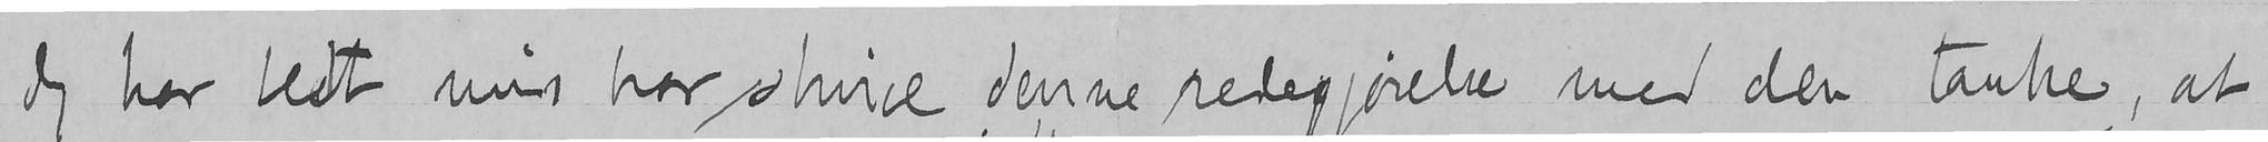Jeg har bedt min bror skrive denne redegjørelse med den tanke, at
